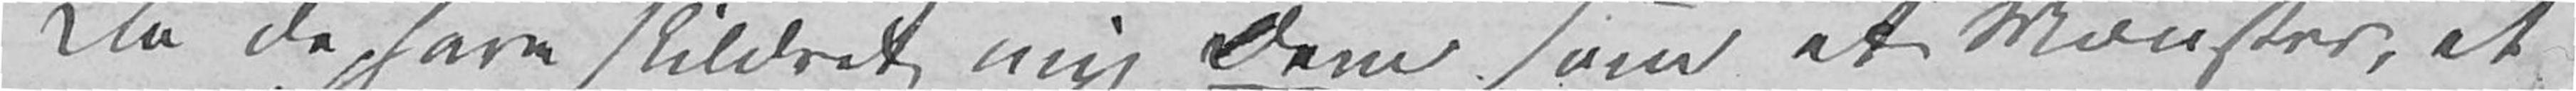Da de have skildret mig Dem som et Mønster, et
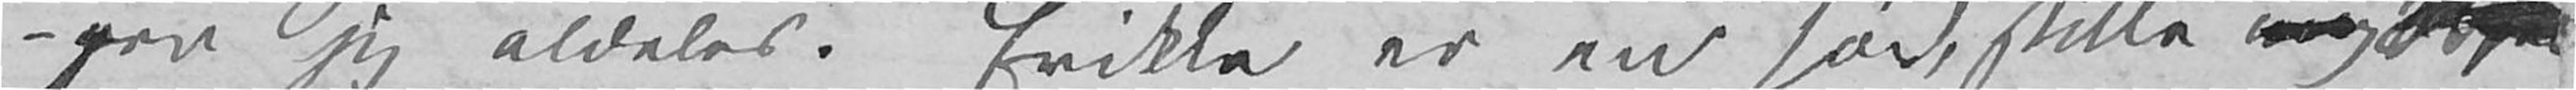ger jeg aldeles. Erikka er en sød, stille <gap /></del>
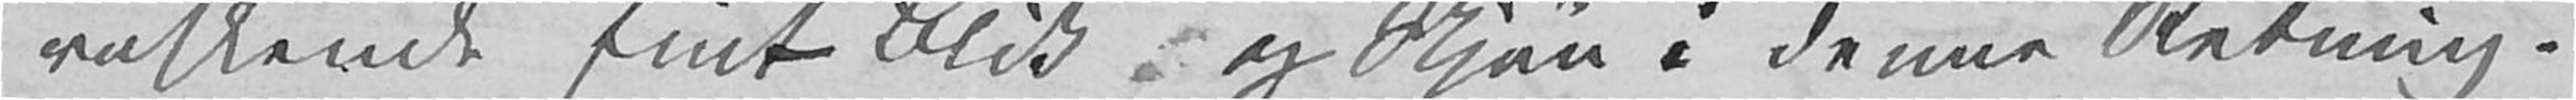raskende fint Blik og Skjøn i denne Retning.
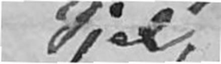Sjel,
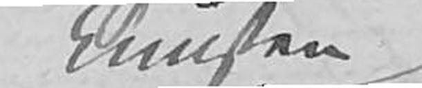Kunsten
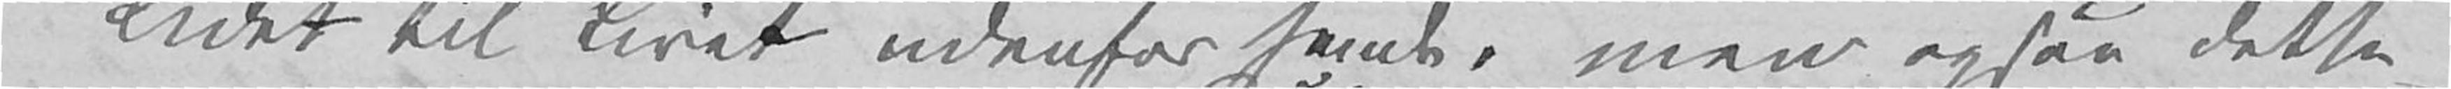lidet til Livet udenfor hende, men ogsaa dette
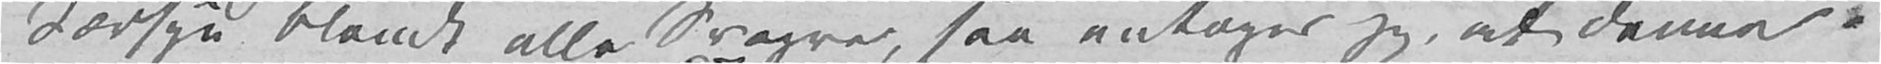Særsyn blandt alle Svogre, saa antager jeg, at denne
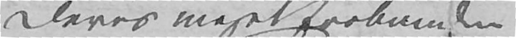Deres meget forbundne
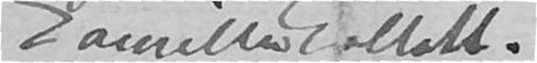Camilla Collett.
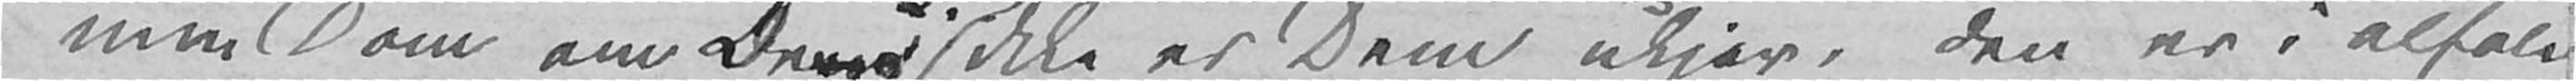min Dom om Deres ikke er Dem ukjer, den er i alfald
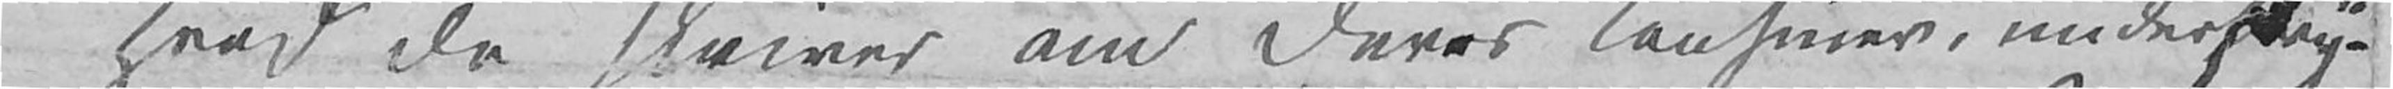Hvad De skriver om Deres Cousiner, understry=
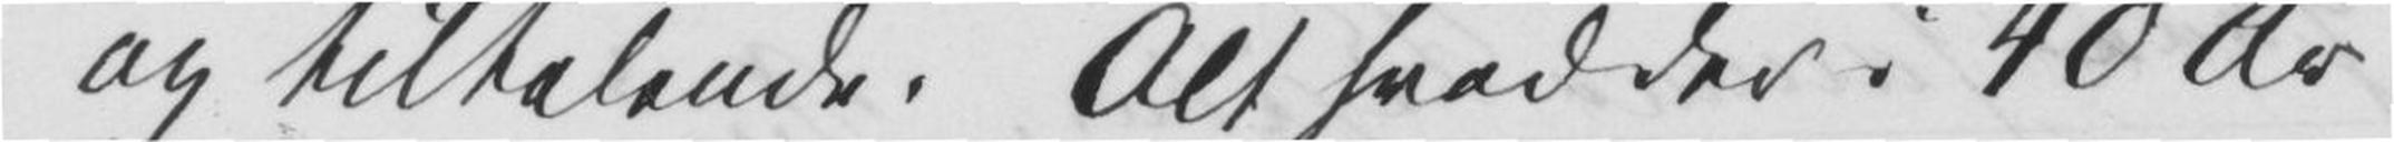og tiltalende. Alt hvad der i 40 År
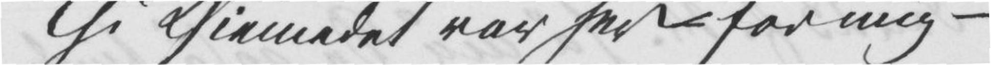Thi Øiemedet var her for mig –
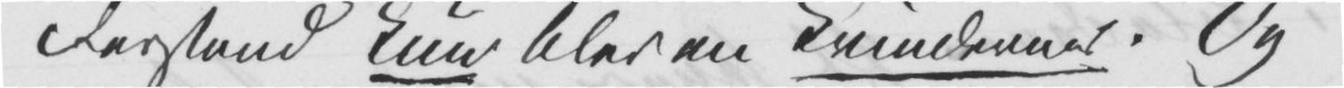Forstand kun blev en Kvindernes. Og
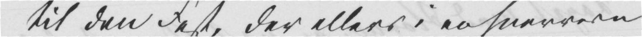til den Fest, der ellers i en snevrere
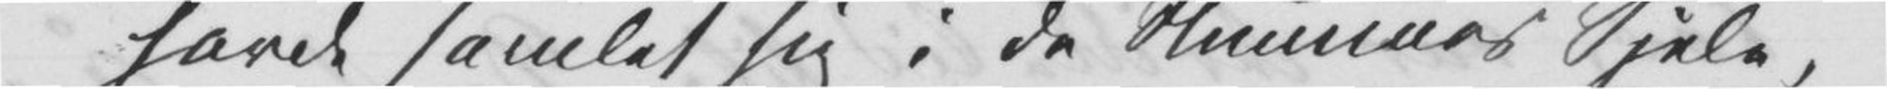havde samlet sig i de Stummes Sjele,
